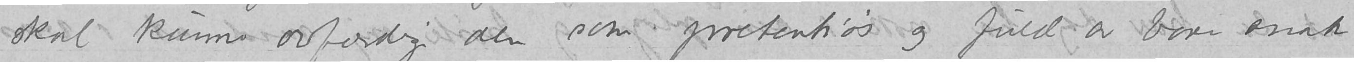skal kunne avfærdige den som pretentiøs og fuld av bare snak
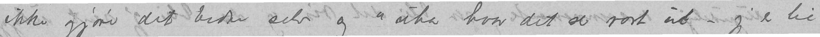ikke gjøre det bedre selv og uha hvor det ser rart ut – jeg er lie
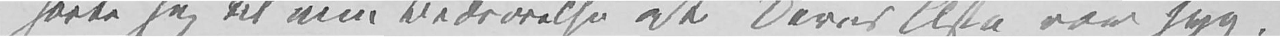hørte jeg til min Bedrøvelse at Deres Asta var syg,
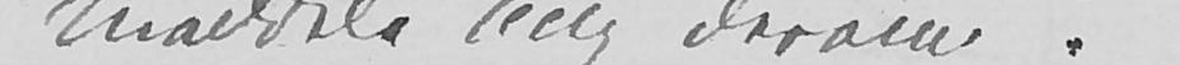meddele mig derom?
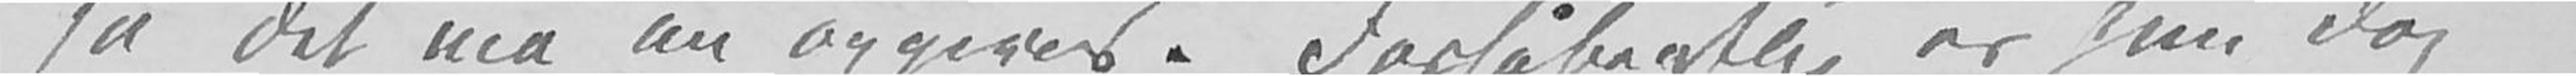så det må nu opgives. Forhåbentlig er hun dog
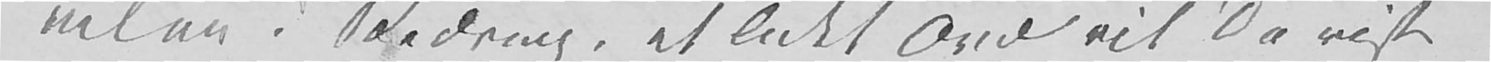vel nu i Bedring, et lidet Ord vil De vist
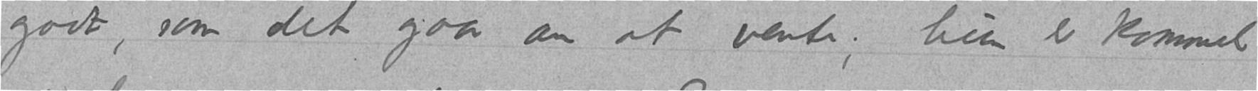godt, som det gaar an at vente; hun er kommel
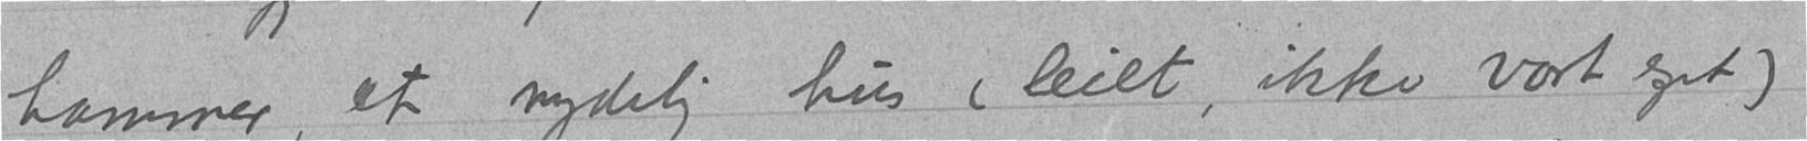hammer, et nydelig hus (leiet, ikke vort eget)
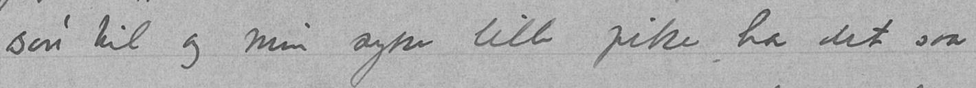søn til og min syke lille pike har det saa
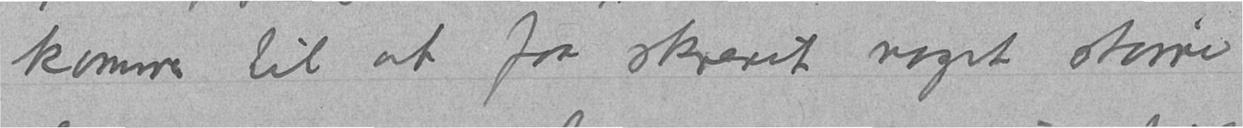kommer til at faa skrevet noget større
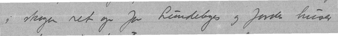i skogen ret op for Lundebyes og Jordes huser
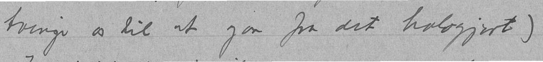tvinge os til at gaa fra det halvgjort)
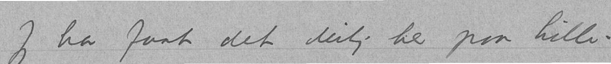Jeg har faat det deilig her paa Lille-
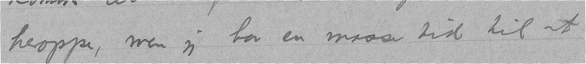heroppe, men jeg har en masse tid til at
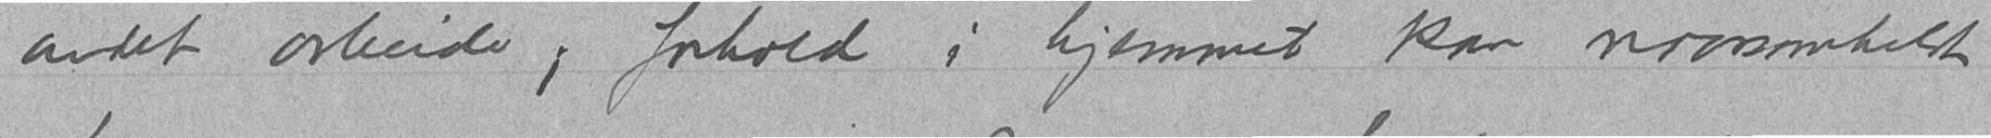andet arbeide, forhold i hjemmet kan naarsomhelst
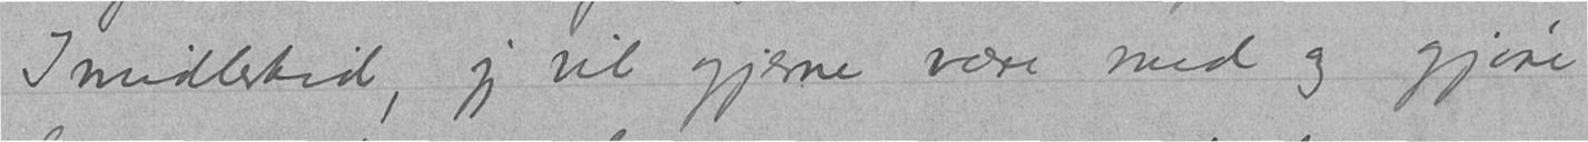Imidlertid, jeg vil gjerne være med og gjøre
